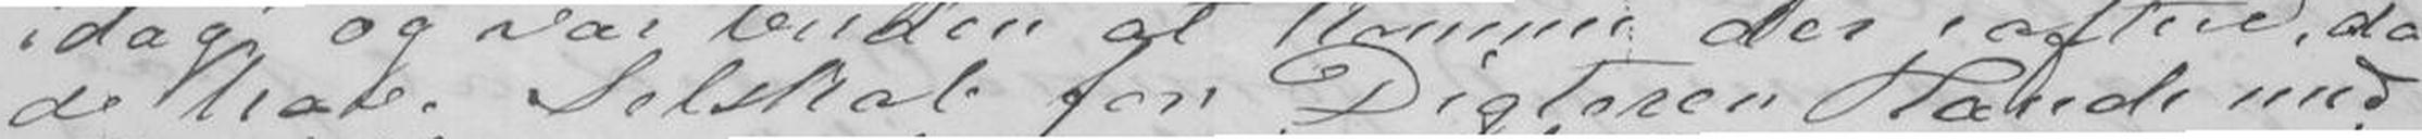de have Selskab for Digteren Hande med
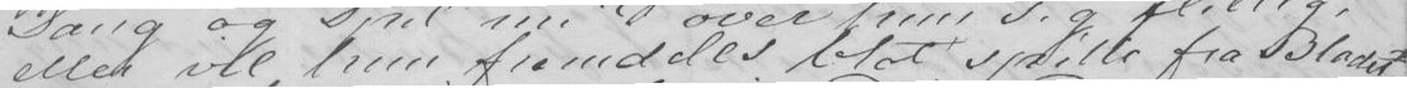eller vil hun fremdeles blot spille fra Bladet.
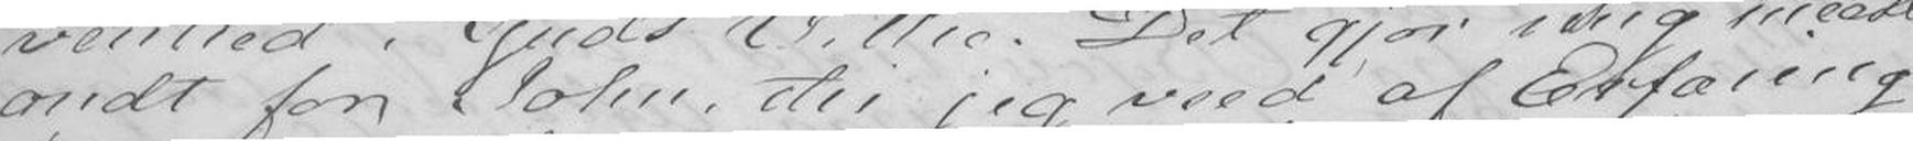ondt for John, thi jeg veed af Erfaring
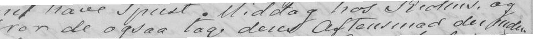rer de ogsaa tage deres Aftensmad der inden
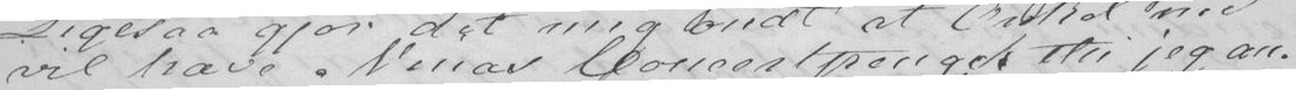vil have Ninas Concertpenge, thi jeg an-
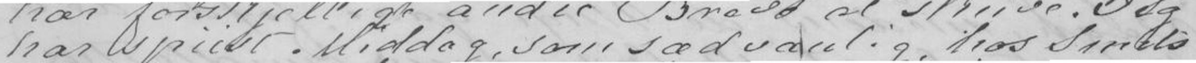har spiist Middag, som sædvanlig hos Smits
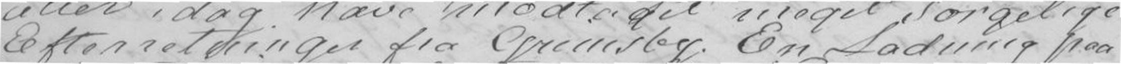Efterretninger fra Grimsby. En Ladning paa
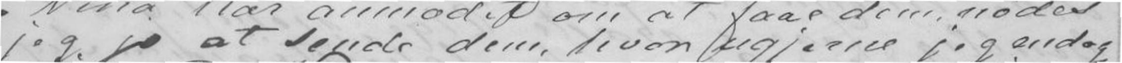jeg jo at sende dem, hvor ugjerne jeg endog
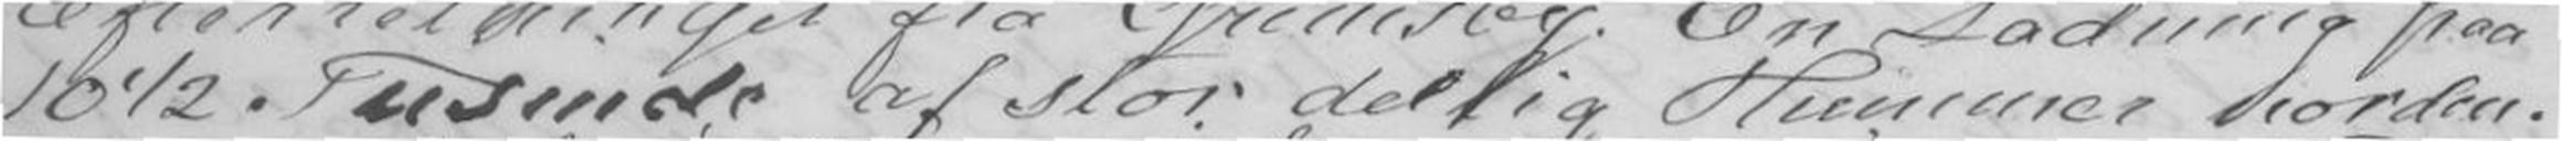!0½ Tusinde af stor deilig Hummer norden-
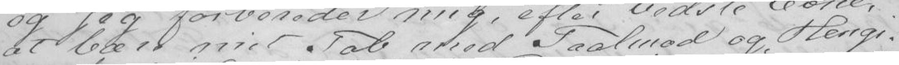at bære mit Tab med Taalmod og Hengi-
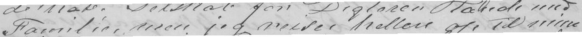Familie, men jeg reiser hellere op til mine
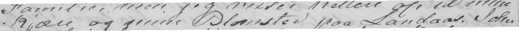kjære og mine Blomster paa Landaas. John
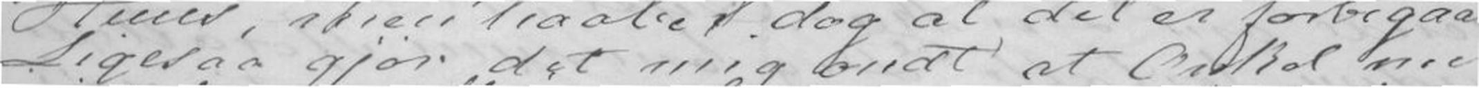Ligesaa gjør det mig ondt at Onkel nu
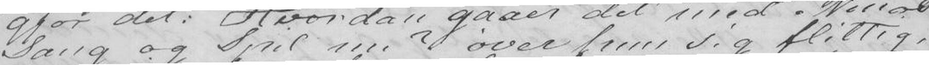Sang og Spil nu? øver hun sig flittig,
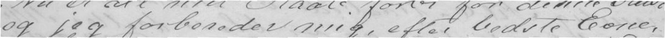og jeg forbereder mig efter bedste Evne
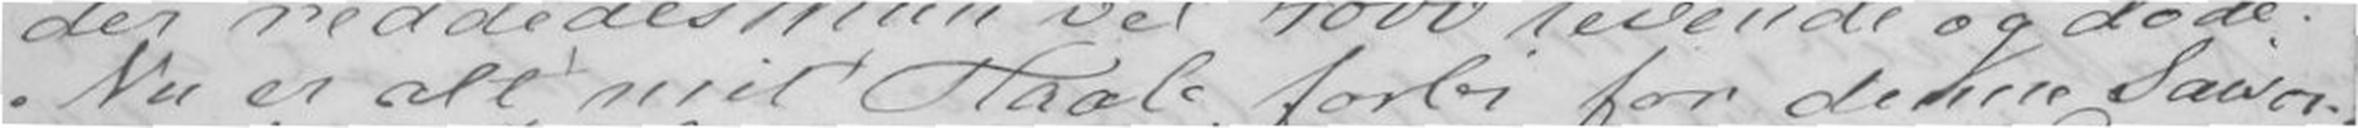Nu er alt mit Haab forbi for denne Saison,
